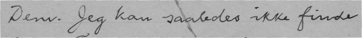Dem. Jeg kan saaledes ikke finde
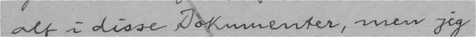alt i disse Dokumenter, men jeg
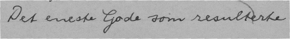Det eneste Gode som resulterte
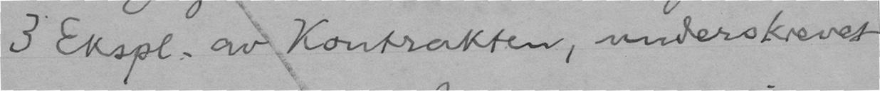3 Ekspl. av Kontrakten, underskrevet
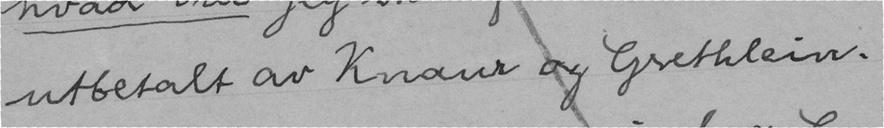utbetalt av Knaur og Grethlein.
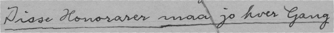Disse Honorarer maa jo hver Gang
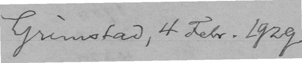Grimstad, 4 Febr. 1929
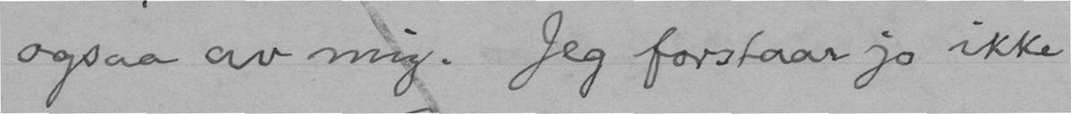ogsaa av mig. Jeg forstaar jo ikke
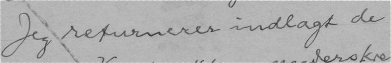Jeg returnerer indlagt de
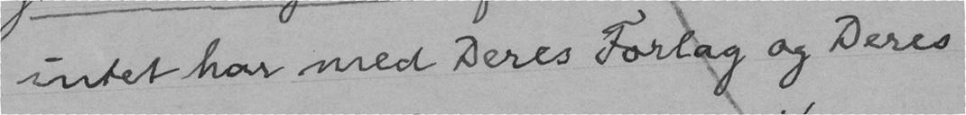intet har med Deres Forlag og Deres
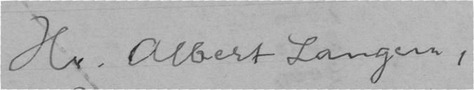Hr. Albert Langen,
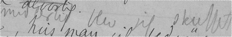imidlertid blev jeg skuffet
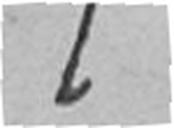1
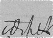værket
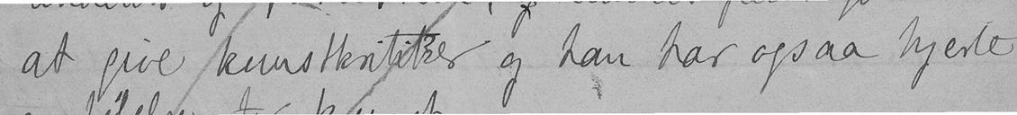at give kunstkritiker og han har ogsaa hjerte
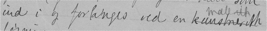ind i og forlanges ved en kunstnerisk
